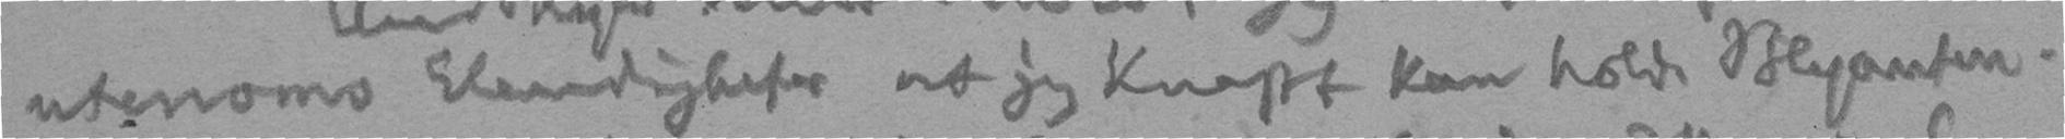utenoms Elendigheter at jeg knapt kan holde Blyanten.
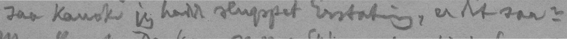saa kanske jeg hadde sluppet Erstatning, er det saa?
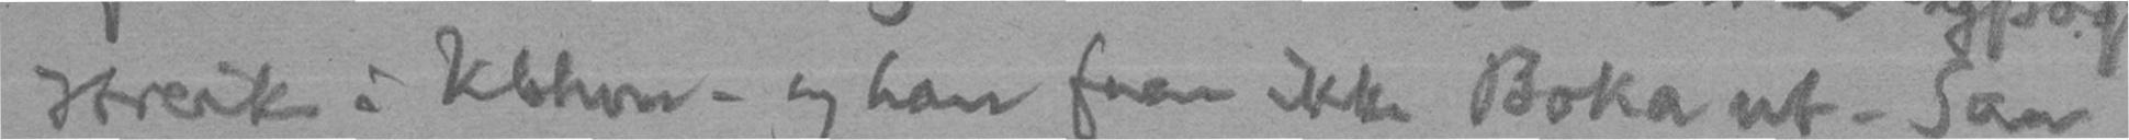streik i Kbhvn. og han faar ikke Boka ut. Saa
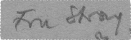Fru Stray
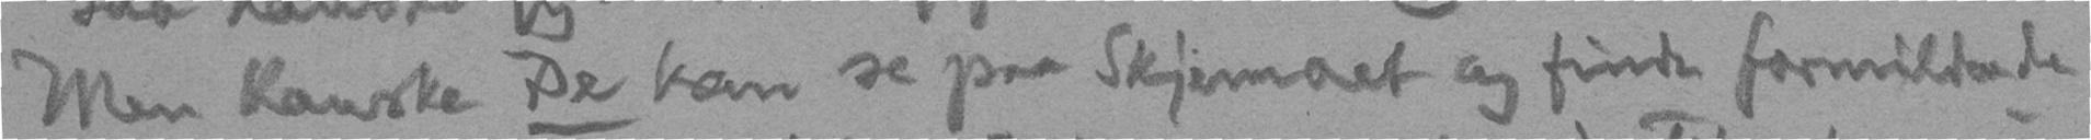Men kanske De kan se paa Skjemaet og finde formildende
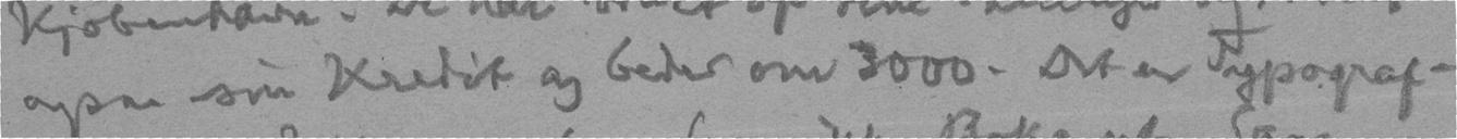ogsaa sin Kredit og beder om 3000. Det en Typograf-
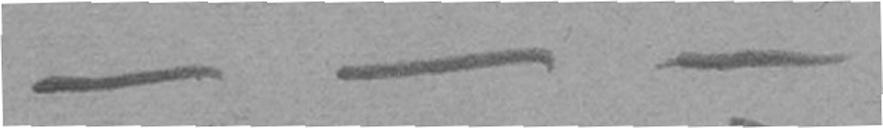- - -
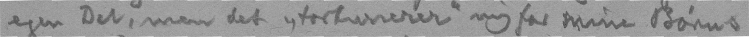egen Del, men det "torturerer" mig for mine Børns
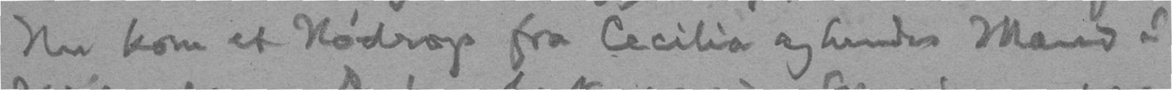Nu kom et Nødrop fra Cecilia og hendes Mand i
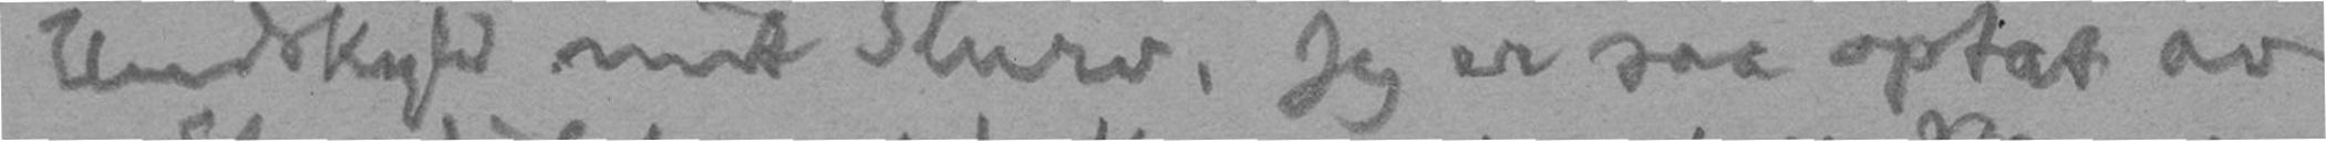Undskyld mit Slurv. Jeg er saa optat av
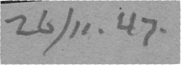26/11. 47.
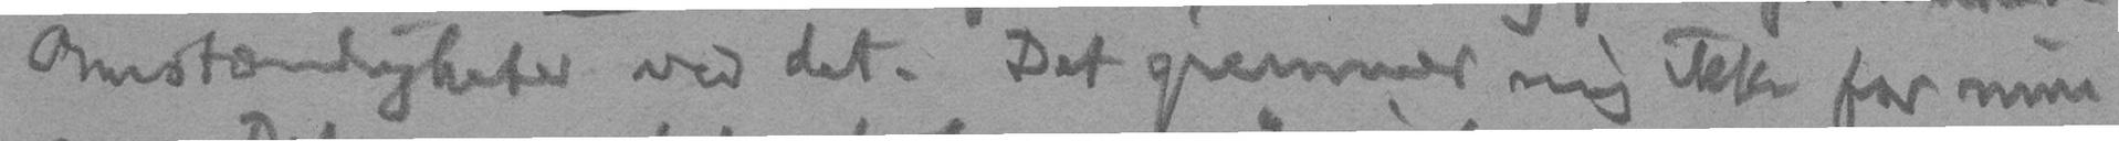Omstændigheter ved det. Det gremmer mig ikke for min
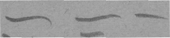- - -
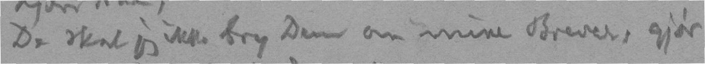De skal jeg ikke bry Dem om mine Brever, gjør
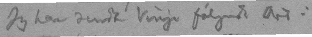Jeg har sendt Vinje følgende Ord:
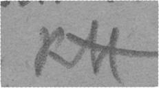KH
